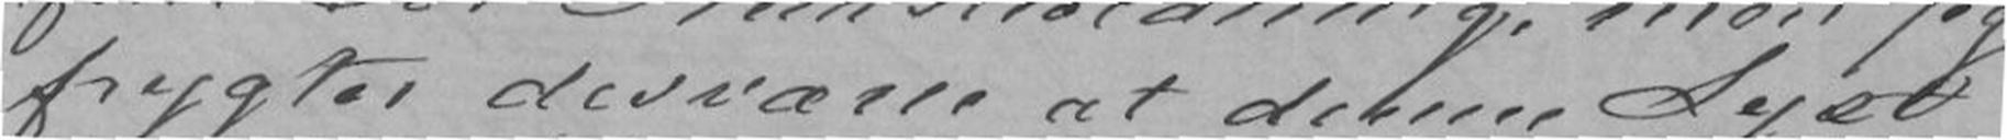frygter desværre at denne Lyst
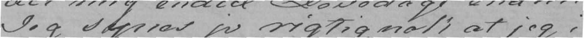Jeg synes jo rigtignok at jeg i
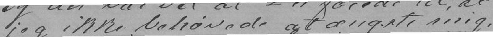jeg ikke behøvede at ængste mig,
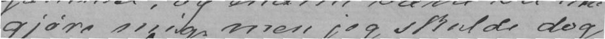gjøre mig, men jeg skulde dog
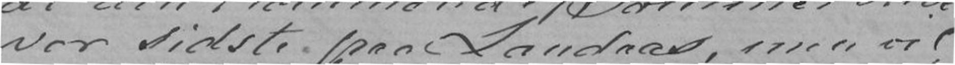vor sidste paa Landaas, men vil
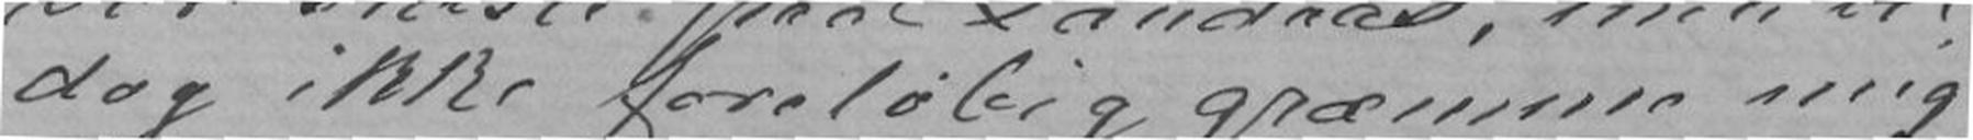dog ikke foreløbig græmmes mig
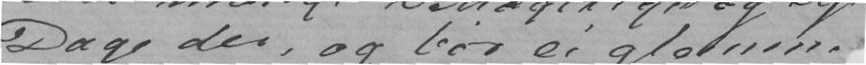Dage der, og bør ei glemme
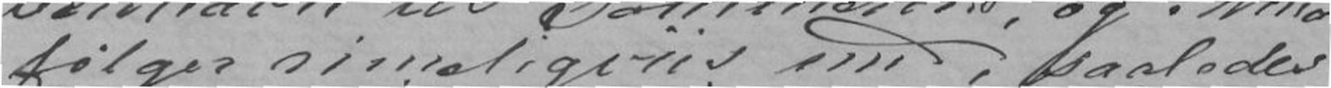følger rimeligviis med, saaledes
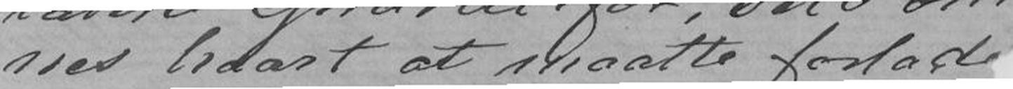nes haart at maatte forlade
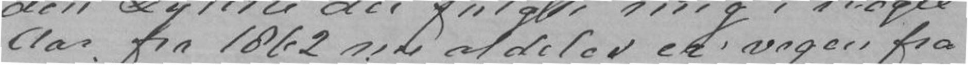Aar fra 1862 nu aldeles er' vegen fra
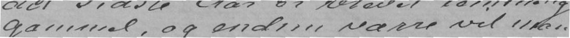gammel, og endnu værre vil man
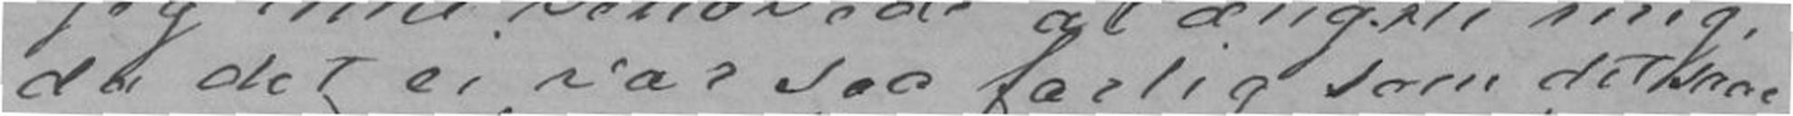da det ei var saa farligt som det saae
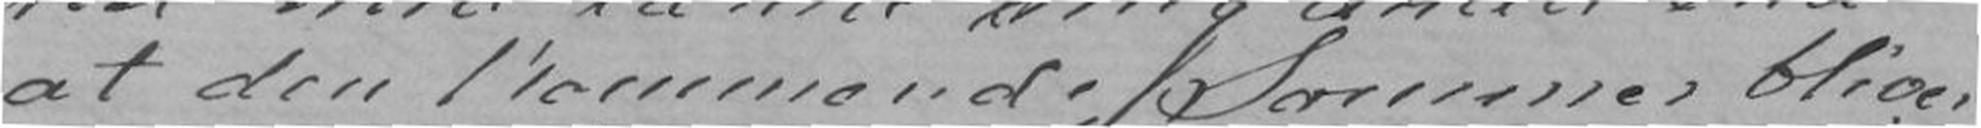at den kommende Sommer bliver
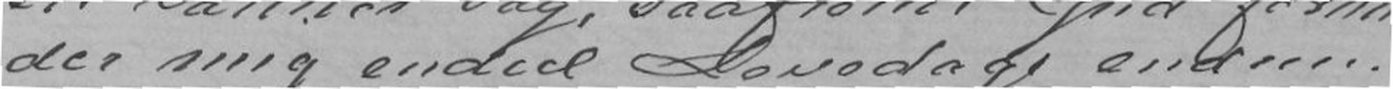der mig endeel Levedags endnu.
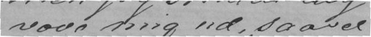vove mig ud, saavel
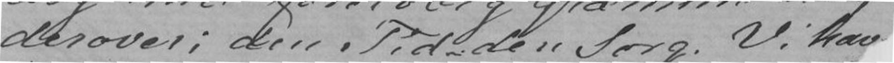derover; den Tid - den Sorg. Vi hav
